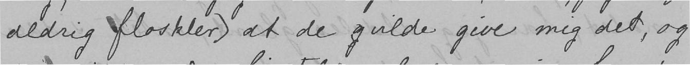aldrig floskler) at de g vilde give mig det, og
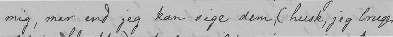mig, mer end jeg kan sige dem, (husk, jeg bruger
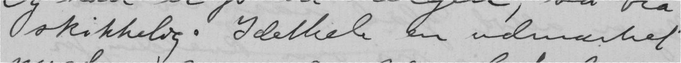skikkelig. I dethele en udmærket
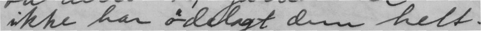ikke har ødelagt dem helt.
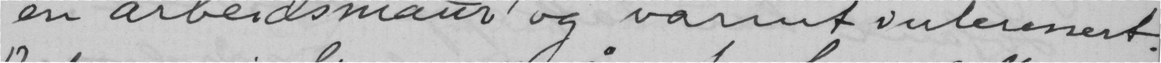en arbeidsmaur og varmt interessert.
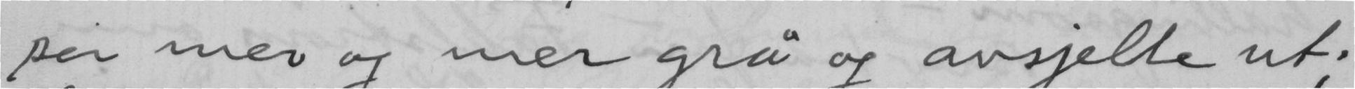ser mer og mer grå og avsjelle ute;
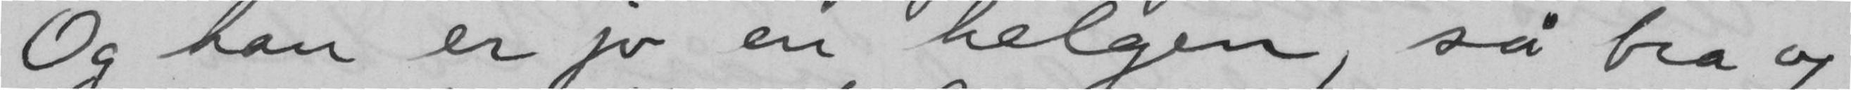Og han er jo en helgen, så bra og
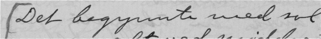Det begynnte med sol
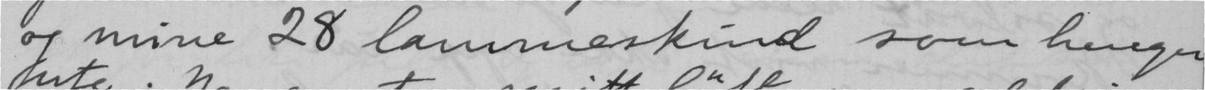og mine 28 lammeskind som henger
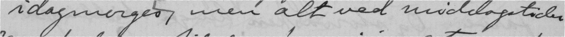idagmorges; men alt ved middagstider
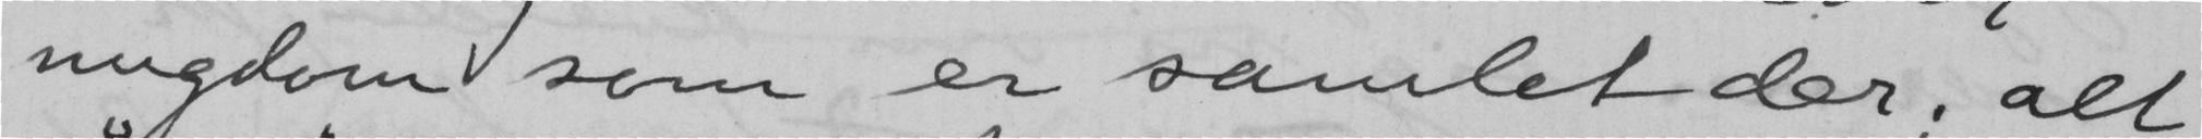ungdom som er samlet der; alt
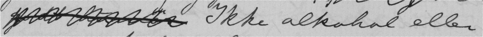Ikke alkohol eller
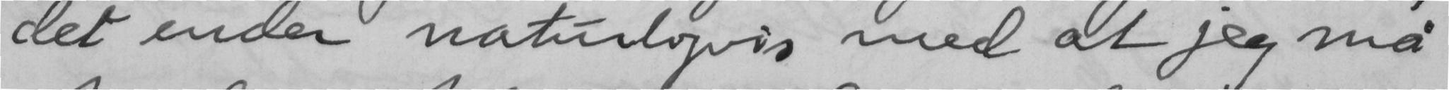det ender naturligvis med at jeg må
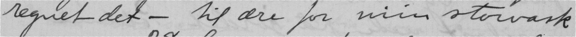regnet det - til ære for min storvask
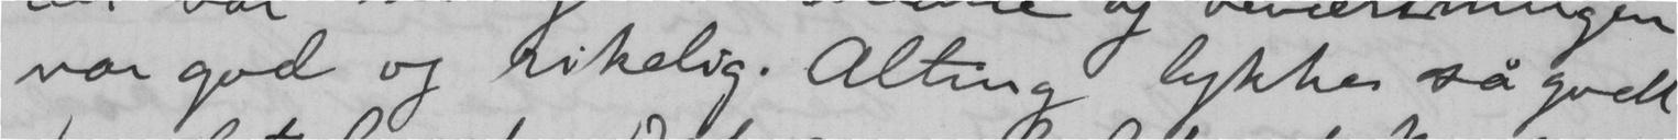var god og rikelig. Alting lykkes så godt
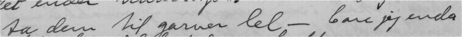ta dem til garver lel - bare jeg enda
